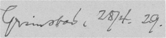Grimstad 28/4. 29.
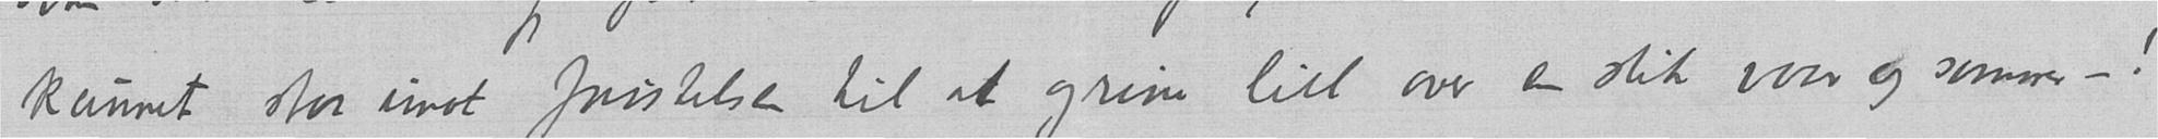kunnet staa imot fristelsen til at grine litt over en slik vaar og sommer – !
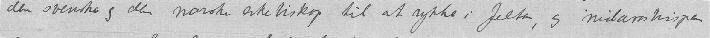den svenske og den norske erkebiskop til at rykke i felten, og nidarosbispen
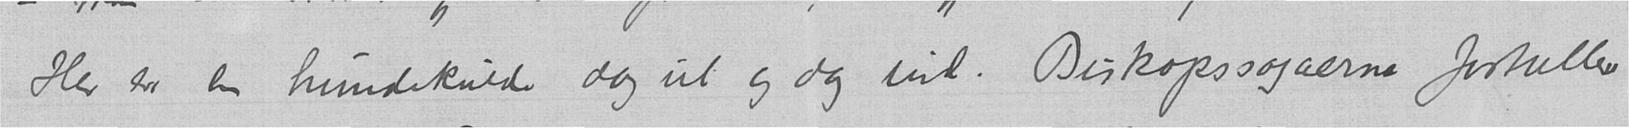Her er en hundekulde dag ut og dag ind. Biskopssagaerne fortæller
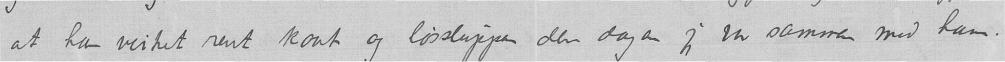at han virket rent kaat og løssluppen den dagen jeg var sammen med ham.
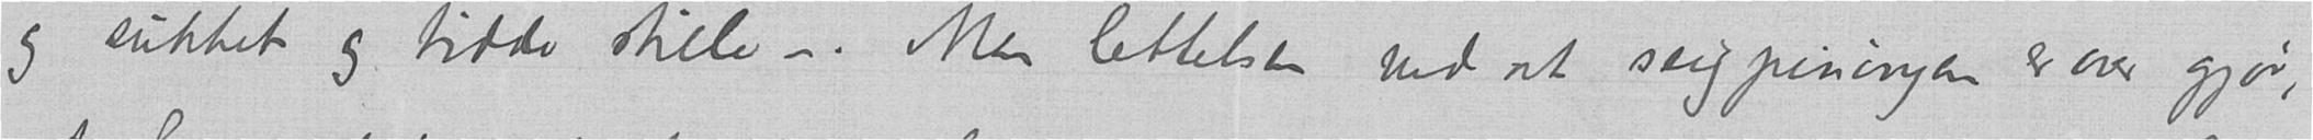og sukket og tidde stille –. Men lettelsen ved at seigpiningen er over gjør,
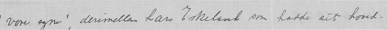"vore egne", derimellem Lars Eskeland som hadde sit hoved-
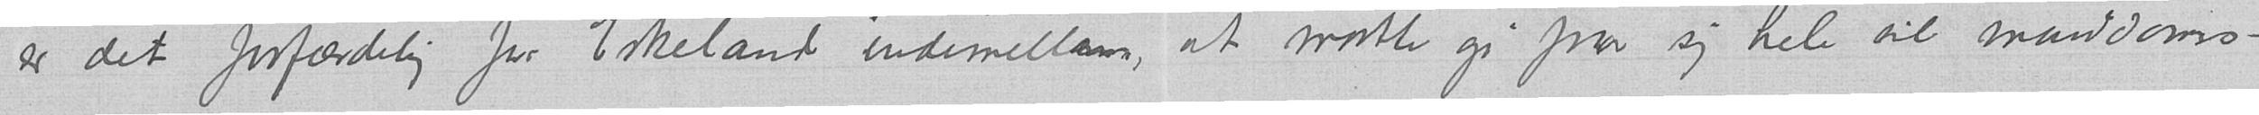er det forfærdelig for Eskeland indimellem, at maatte gi fra sig hele sit manddoms-
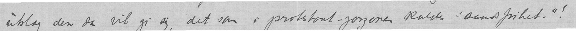utslag den da vil gi sig, det som i protestant-jargonen kaldes "aandsfrihet."!
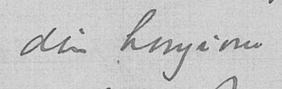din hengivne
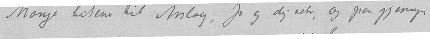Mange hilsener til Aaslaug, Jo og dig selv, og paa gjensyn
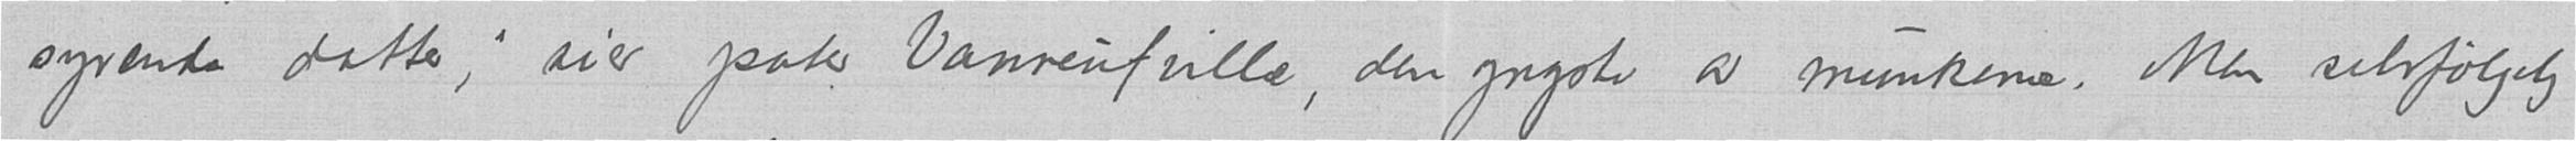syvende datter", sier pater Vanneufville, den yngste av munkene. Men selvfølgelig
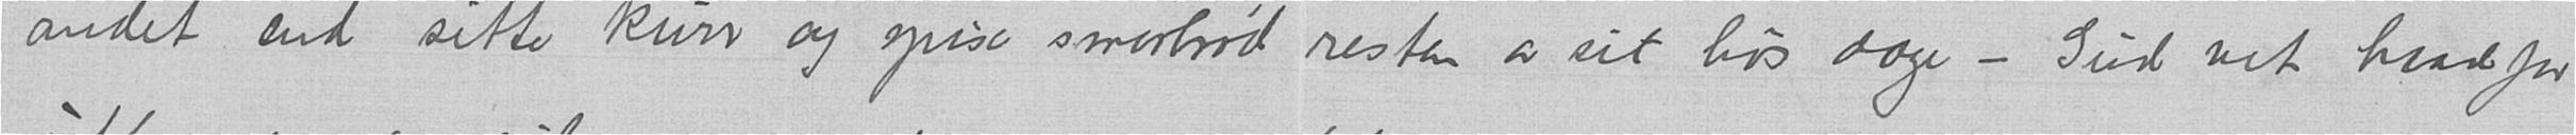andet end sitte kurr og spise smørbrød resten av sit livs dage – Gud vet hvadfor
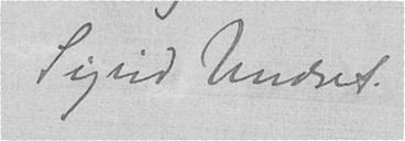Sigrid Undset.
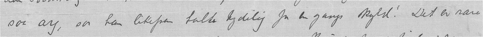saa arg, saa han likefrem talte tydelig for en gangs skyld! Det er rare
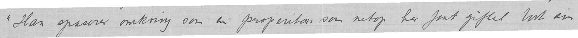"Han spaserer omkring som en properitær som netop her faat giftet bort sin
